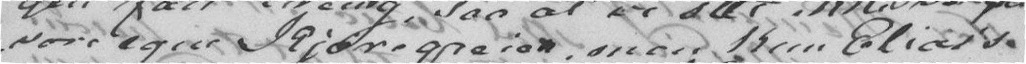vore egne Kjøregreier, men kun Elias's
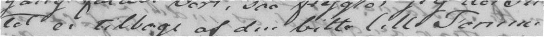tet er tilbage af den bitte lille Formue
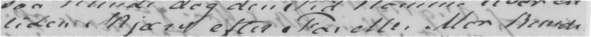liden Skjærv efter Far eller Mor kunde
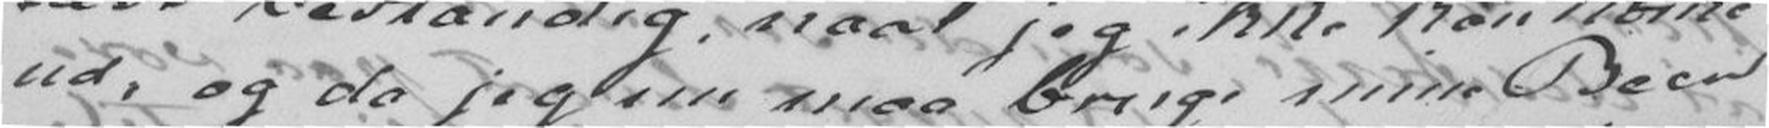ud, og da jeg nu maa bruge mine Been
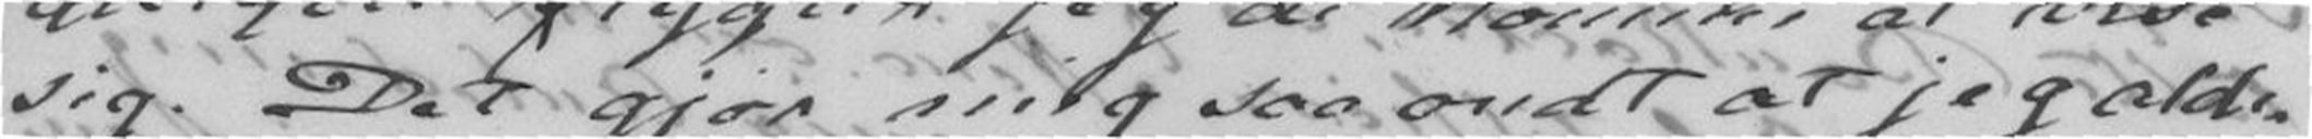sig. Det gjør mig saa ondt at jeg alde-
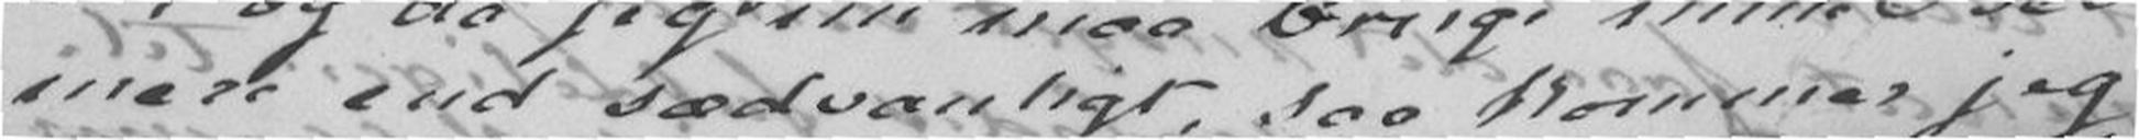mere end sædvanligt, saa kommer jeg
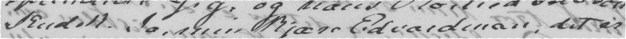Kudsk. Ja, min kjære Edvardman, det er
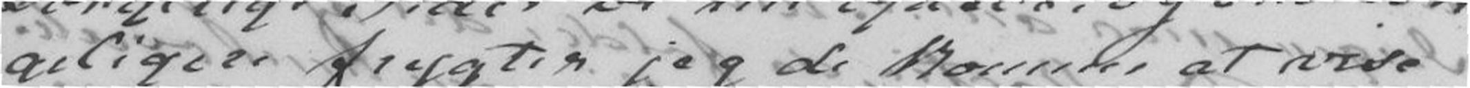geligere frygter jeg de komme at vise
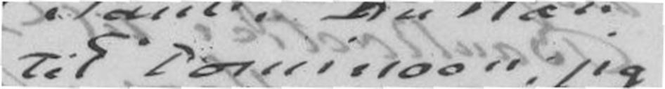til Dominoen, jeg
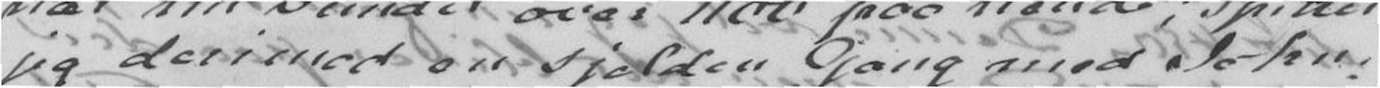jeg derimod en sjelden Gang med John;
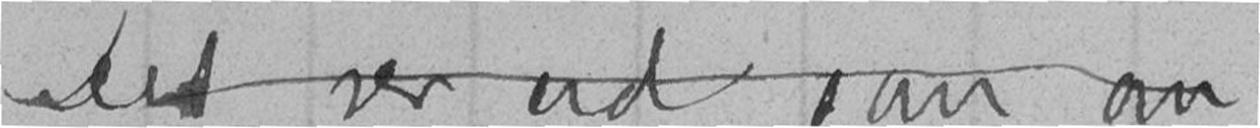Det ser ud som om
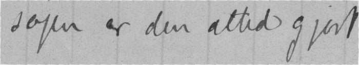sagen er den altid gjort
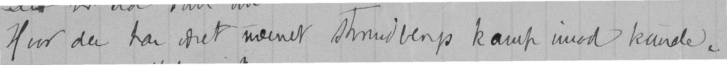Hvor der har været nævnet Strindbergs kamp imod kunde i
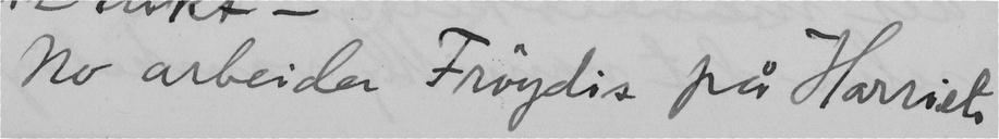No arbeidar Fröydis på Harriets
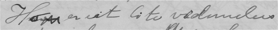Hun er eit lite vidunderleg
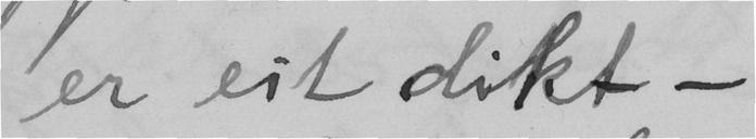er eit dikt –
